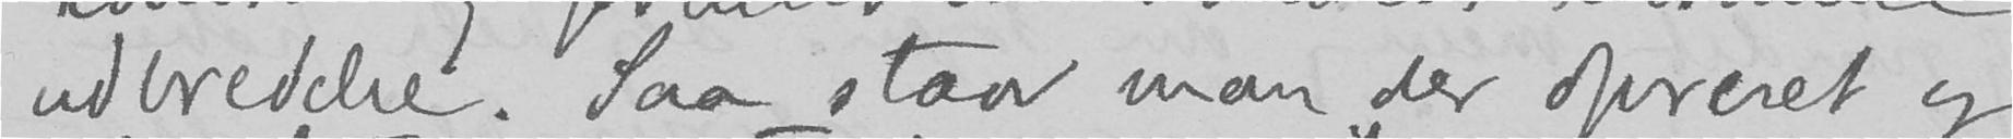udbredelse. Saa staar man der oprevet og
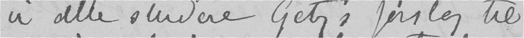vi alle studere Getzs forslag til
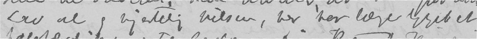Lev vel og hjertelig hilsen, her har længe ligget et
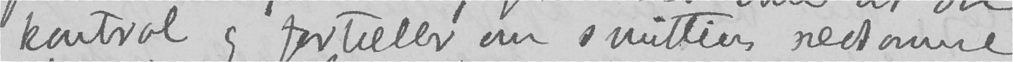kontrol og fortæller om smittens redsomme
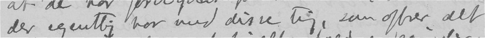der egentlig har med disse ting, som offrer alt
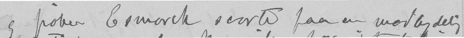og frøken Esmarck svarte paa en modbydelig
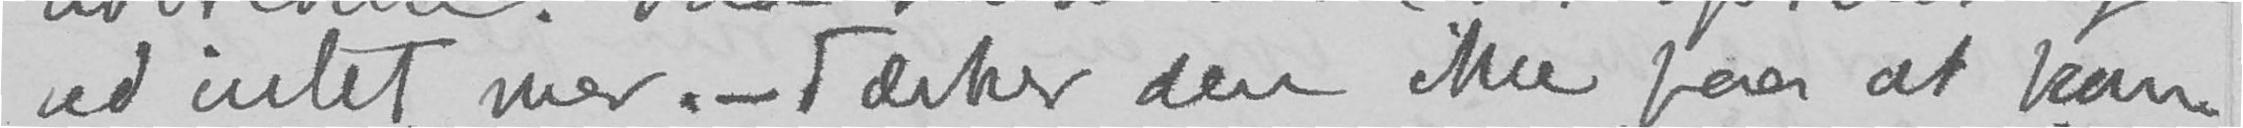ved intet mer. - Tænker dere ikke paa at kom-
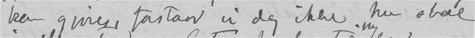kan gjøres, forstaar vi dog ikke. Nu skal
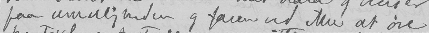paa umuligheden og faren ved ikke at øve
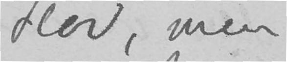lov, men
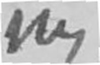ny
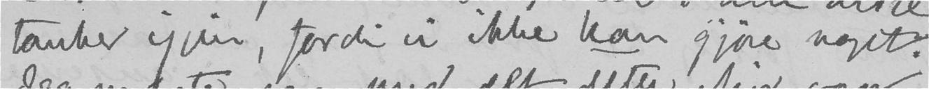tanker igjen, fordi vi ikke kan gjøre noget.
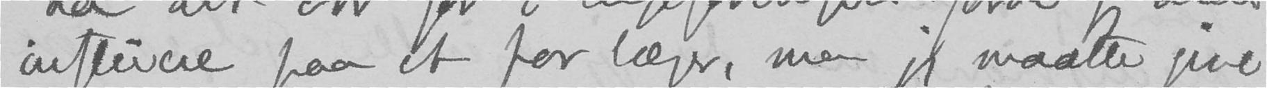influere paa et par læger, men jeg maatte give
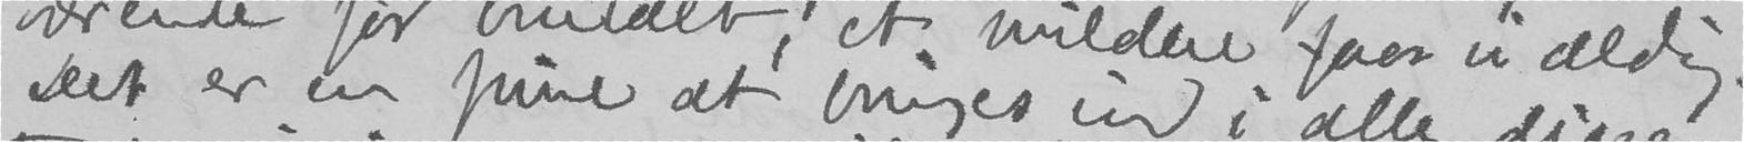værende for brutalt, et mildere faar vi aldrig.
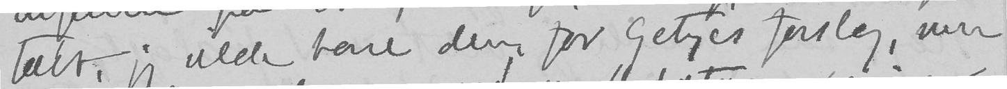tabt, jeg vilde have dem for Getzes forslag, men
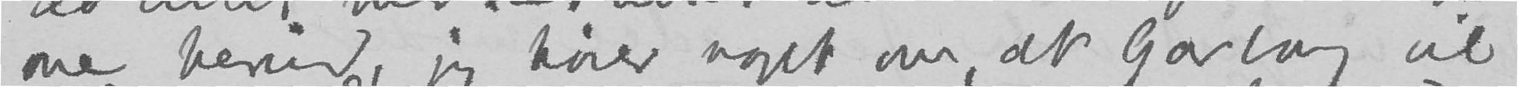me herind, jeg hører noget om, at Garborg vil
